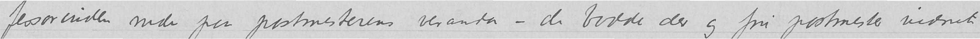fessorinden nede paa postmesterens veranda - de bodde der og fru postmester vidnet
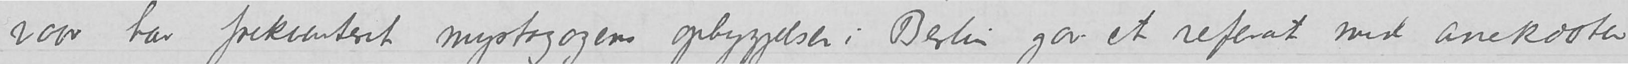vaar har frekventert mystagogens opbyggelse i Berlin gav et referat med anekdoter
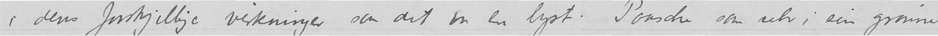i dens forskjellige virkninger saa det var en lyst. Paasche som selv i sin grønne
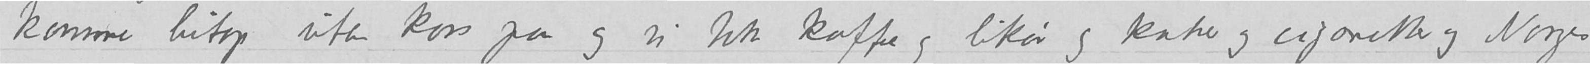komme hitop uten kors paa og vi tok kaffe og likør og kake og cigaretter og Norges
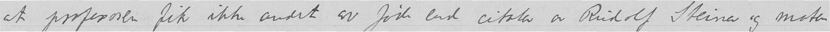at professoren fik ikke andet av føde end citater av Rudolf Steiner og maten
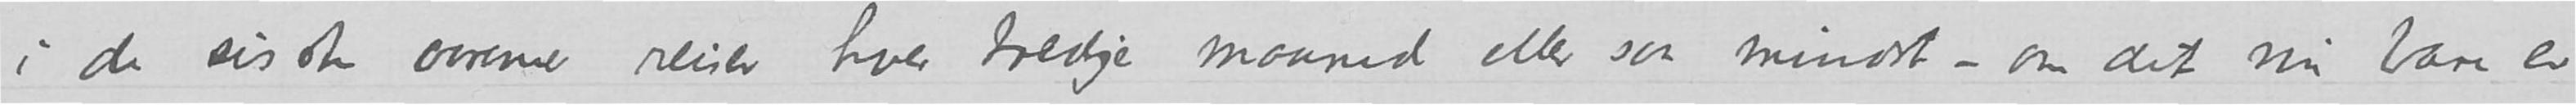i de sisste aarene reiser hver tredje maaned eller saa mindst - om det nu bare er
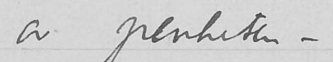av penheten - .
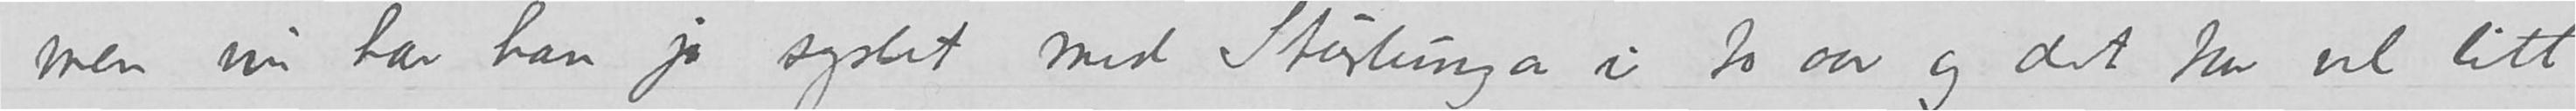mend nu har han jo syslet med Sturlunga i to aar og det tar vel litt
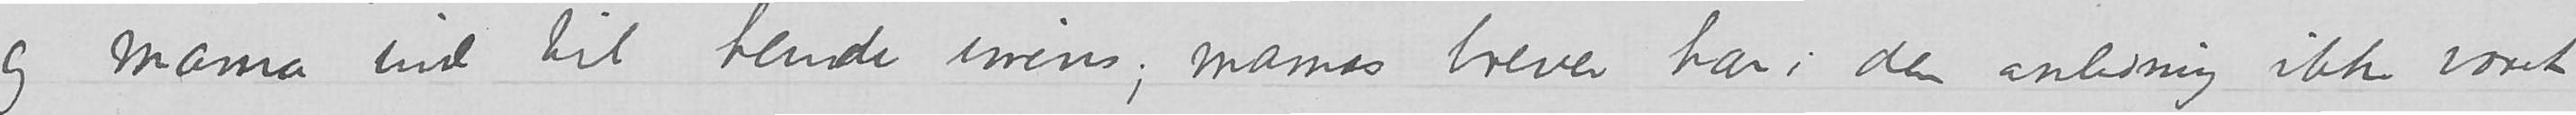og mama ind til hende imens; mamas brever har i den anledning ikke været
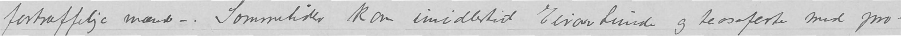fortræffelige mænd -. Sommetider kom imidlertid Einar Lunde og teosoferte med pro-
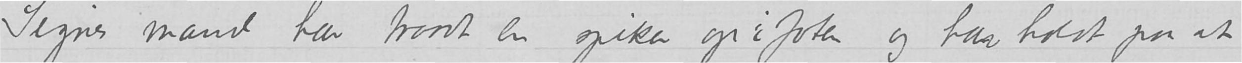Signes mand har traadt en spiker opp i foten og har holdt paa at
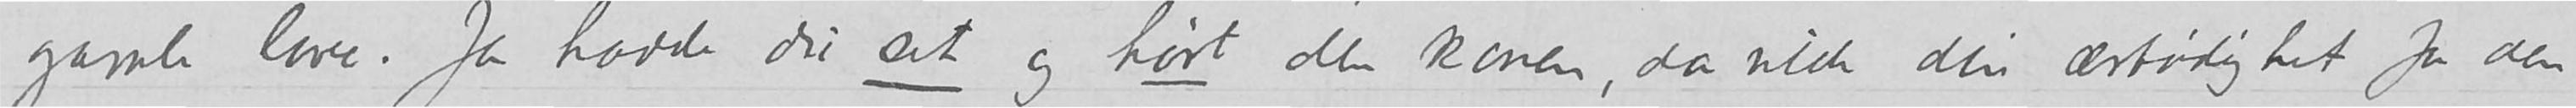gamle love. Ja hadde du set og hørt den konen, da vilde din ærbødighet for den
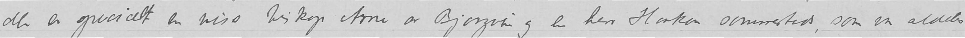det er spesielt en viss biskop Arne av Bjørgvin og en herr Horken sommesteds, som er aldeles
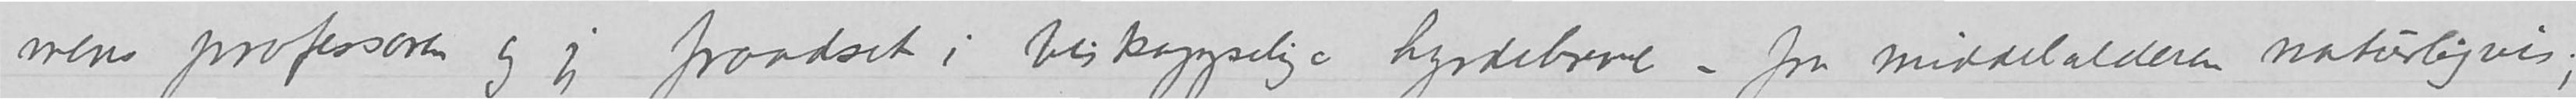mens professoren og jeg fraadset i biskoppelige hyrdebreve - fra middelalderen naturligvis;
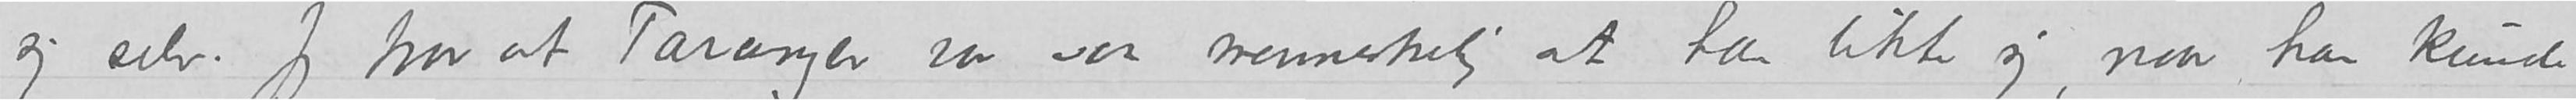sieg selv. Jeg tror at Taranger var saa menneskelig at han likte seg, naar han kunde
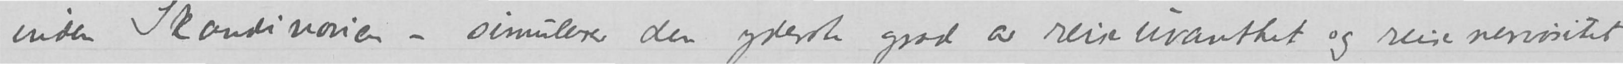inden Skandinavien - simulerer den yderste grad av reiseuvanthet og reisenervøsitet
